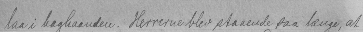laa i baghaanden. Herrerne blev staaende saa længe, at
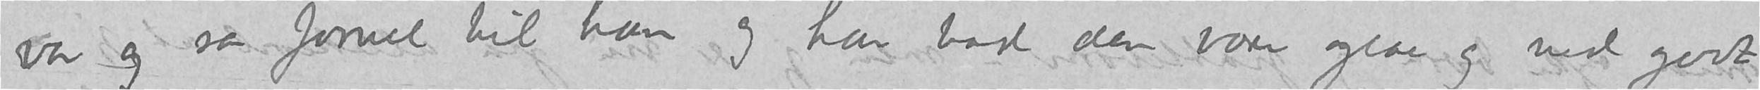var og sa farvel til ham og han bad dem være glae og ved godt
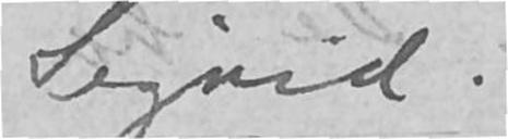Sigrid.
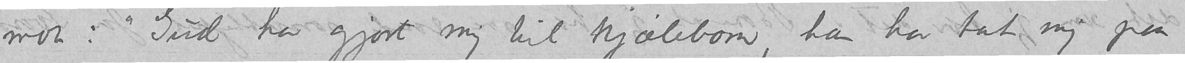mot: «Gud har gjort mig til kjælebarn, han har tat mig paa
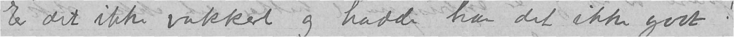Er det ikke vakkert og hadde han det ikke godt!
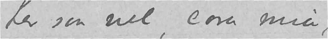Lev saa vel, cora mia,
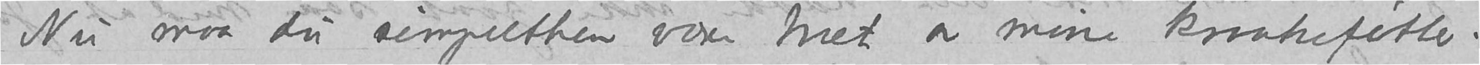Nu maa du simpelthen være træt av mine kraakeføtter.
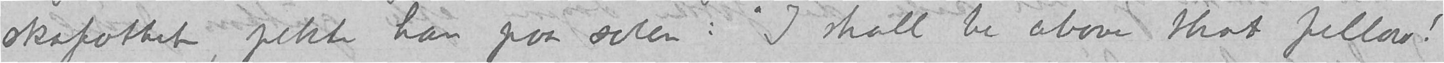skafottet, pekte han paa solen: «I shall be above that fellow!»
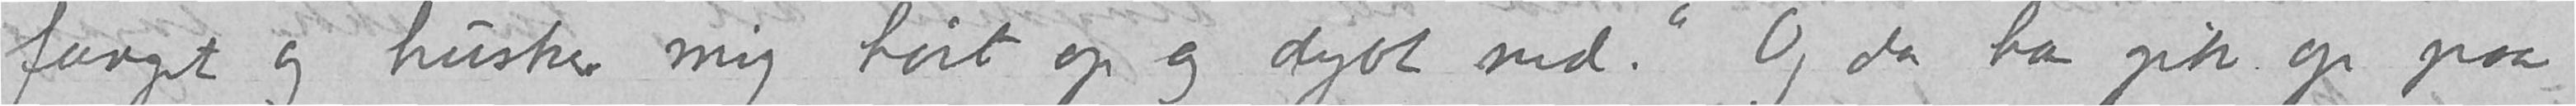fanget og husker mig høit op og dybt ned.» Og da han gik op paa
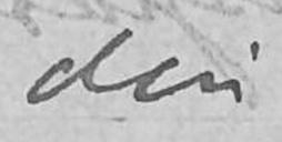din
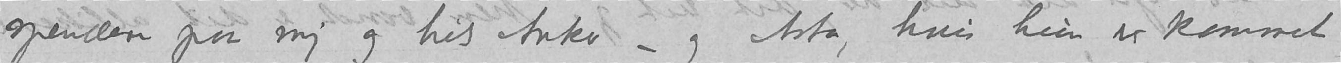spandere paa mig og hils Anker – og Asta, hvis hun er kommet
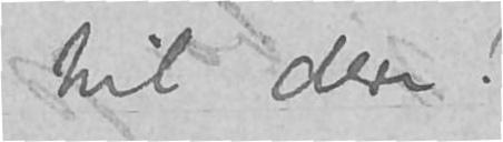til dere!
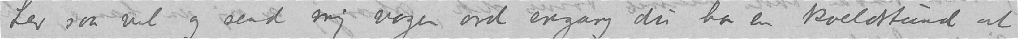Lev saa vel og send mig nogen ord engang du har en kveldstund at
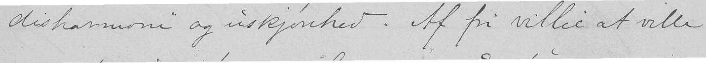disharmoni og uskjønhed. Af fri villie at ville
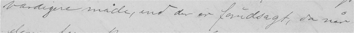værdigere måde, end der er forudsagt, da når
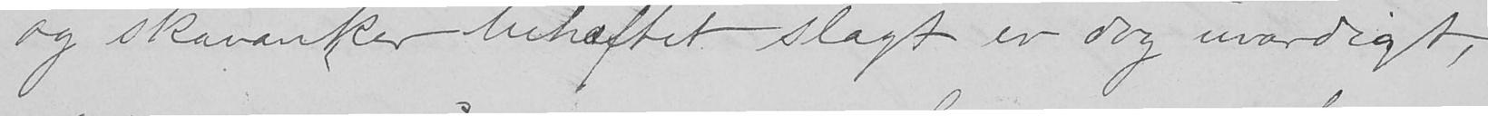og skavanker behæftet slægt er dog uværdigt,
